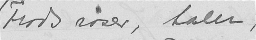Trods roser, taler,
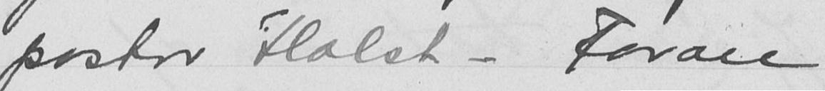pastor Holst. Foran
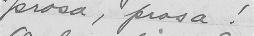prosa, prosa!
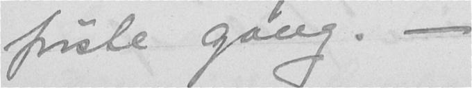første gang. -
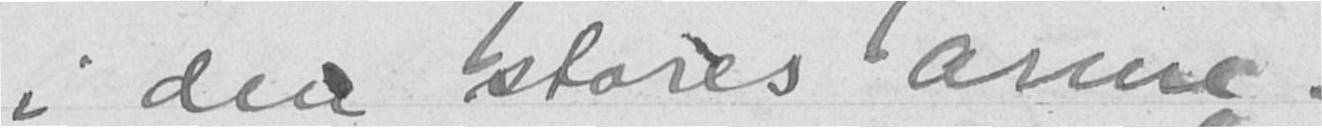i den stores arme.
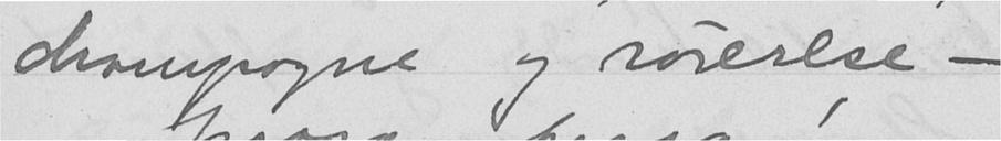champagne og rørerlse -
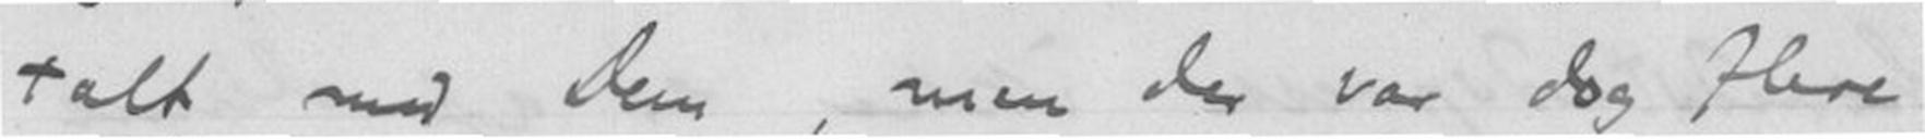talt med Dem, men der var dog flere
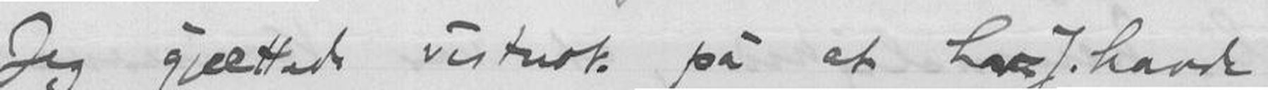Jeg gjættede vistnok på at hr. J. havde
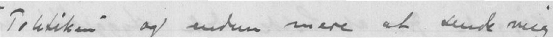"Taktiken" og endnu mere at sende mig
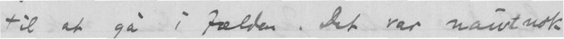til at gå i fælden. Det var naivt nok
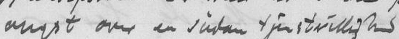angst over en sådan tjenstvillighed.
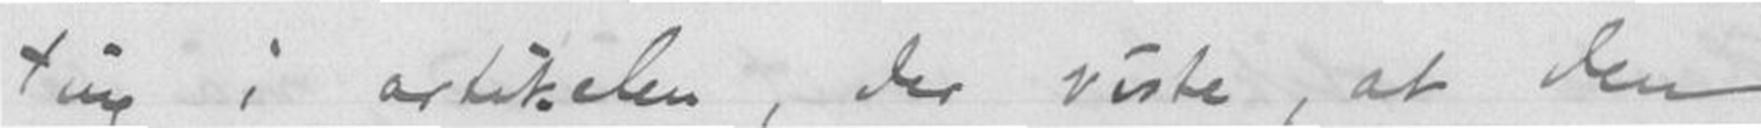ting i artikelen, der viste, at den
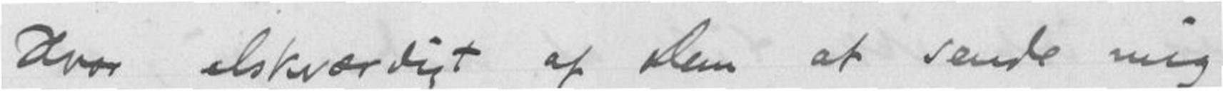Hvor elskværdigt af Dem at sende mig
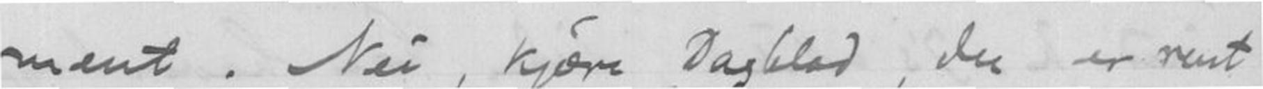ment. Nei, kjære Dagblad, den er rent
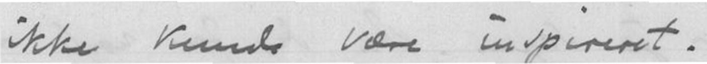ikke kunde være inspireret.
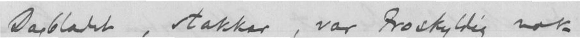Dagbladet, stakker, var troskyldig nok
